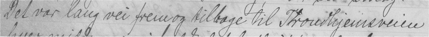Det var lang vei frem og tilbage til Trondhjemsveien
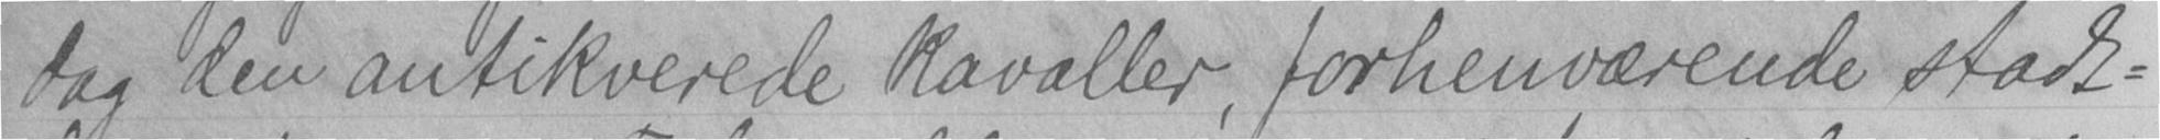dag den antikverede kavaller, forhenværende stadt-
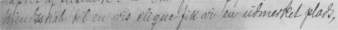kjendtskab til en vis clique fik vi en udmærket plads,
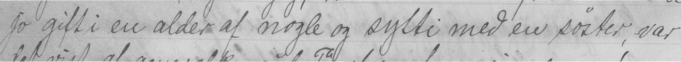jo gift i en alder af nogle og sytti med en søster, var
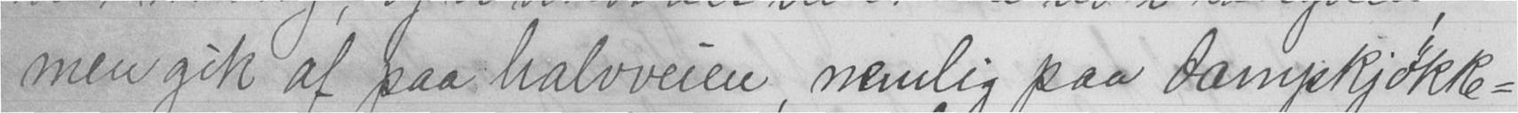men gik af paa halvveien, nemlig paa dampkjøkke-
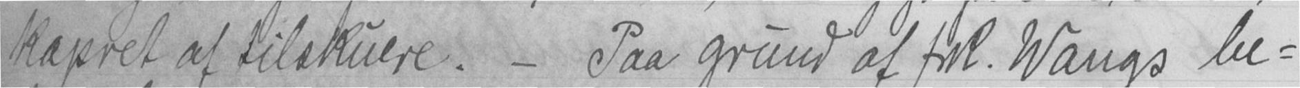kapret af tilskuere. - Paa grund af frk. Wangs be-
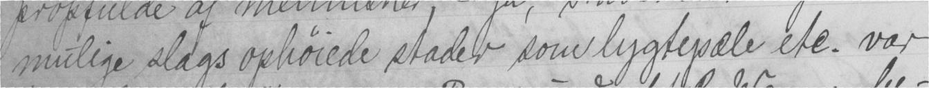mulige slags ophøiede stader som lygtepæle etc. var
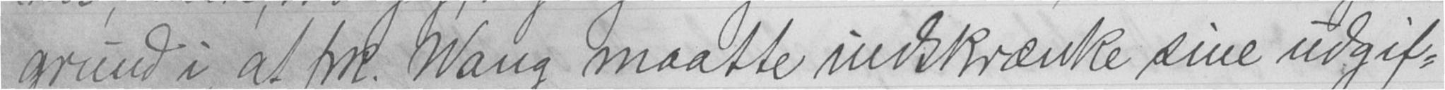grund i, at frk. Wang maatte indskrænke sine udgif-
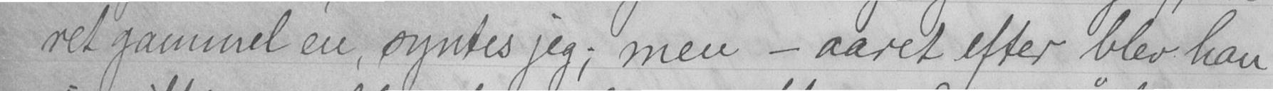ret gammel en, syntes jeg; men - aaret efter blev han
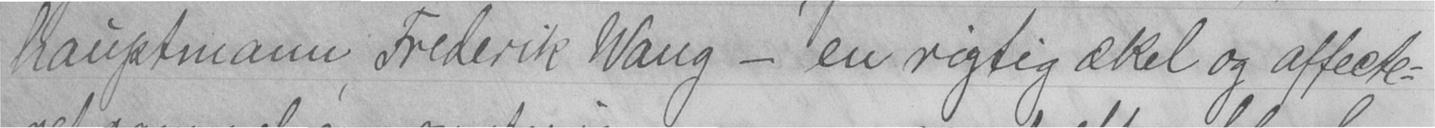hauptmann, Frederik Wang - en rigtig ækel og affecte-
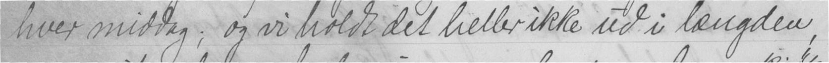hver middag; og vi holdt det heller ikke ud i længden,
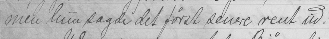men hun sagde det først senere rent ud.
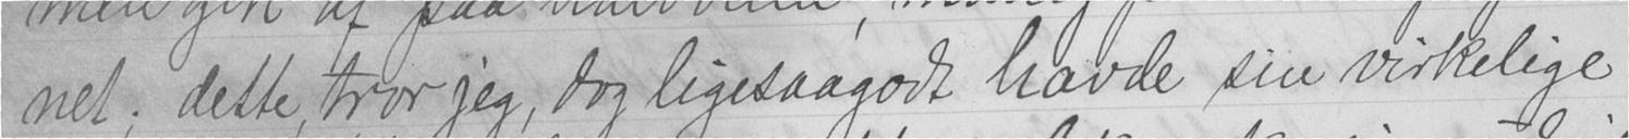net; dette, tror jeg, dog ligesaagodt havde sin virkelige
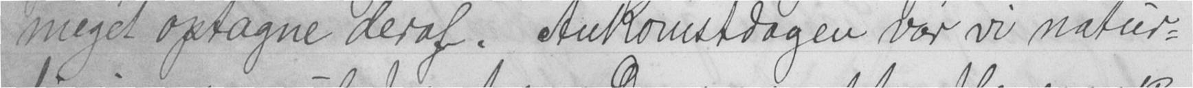meget optagne deraf. Ankomstdagen var vi natur-
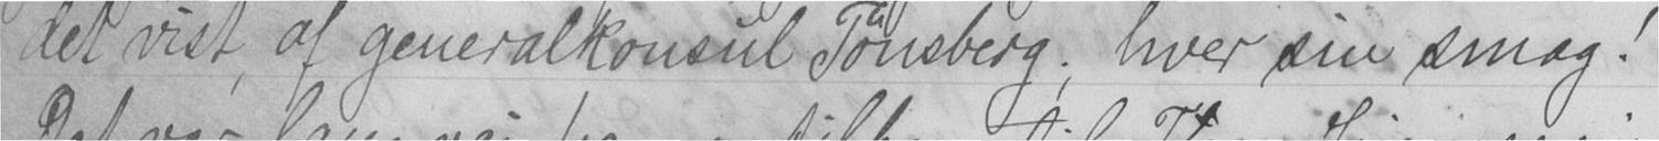det vist, af generalkonsul Tønsberg; hver sin smag!
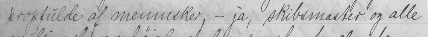propfulde af mennesker, - ja, skibsmaster og alle
